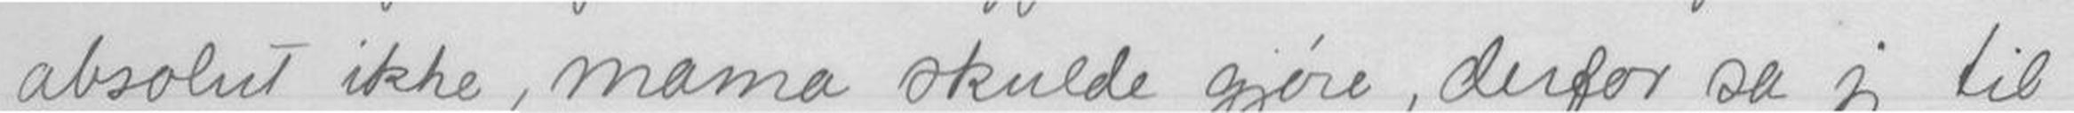absolut ikke, mama skulde gjøre, derfor sa jeg til
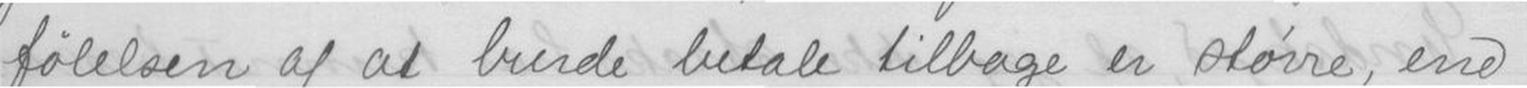følelsen af at burde betale tilbage er større, end
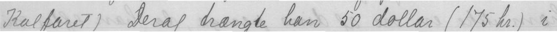Kalfaret). Deraf trængte han 50 dollar (175 kr.) i
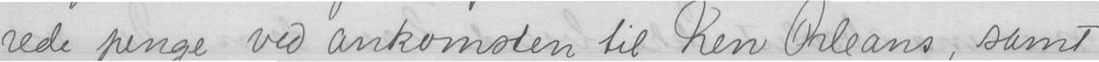rede penge ved ankomsten til New Orleans, samt
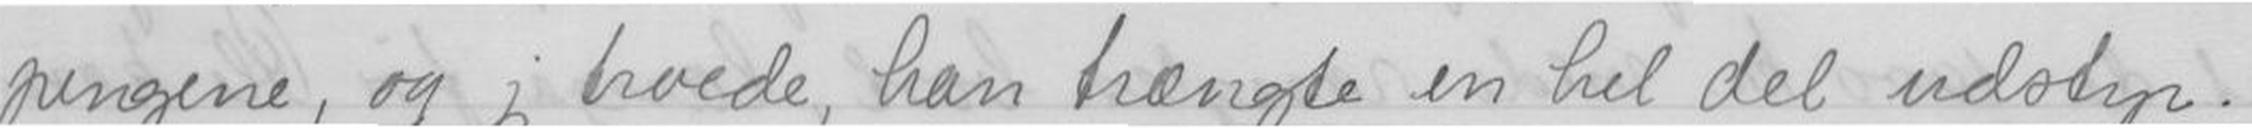pengene, og jeg troede, han trængte en hel del udstyr.
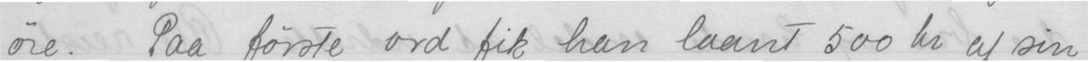øre. Paa første ord fik han laant 500 kr af sin
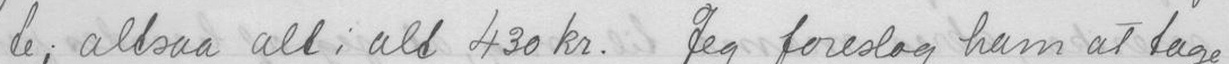te, altsaa alt i alt 430 kr. Jeg foreslog ham at tage
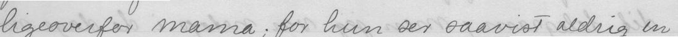ligeoverfor mama; for hun ser saavist aldrig en
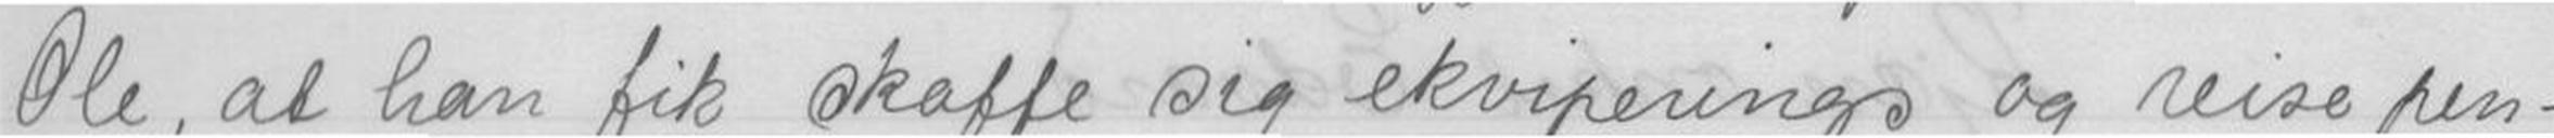Ole, at han fik skaffe sig ekviperings og reisepen-
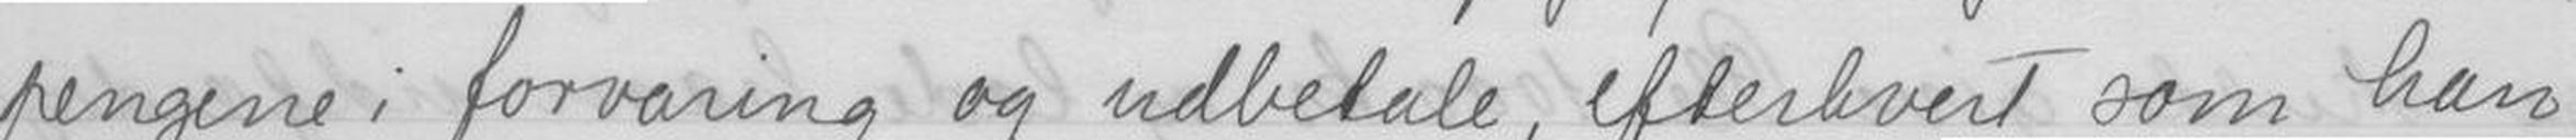pengene i forvaring og udbetale, efterhvert som han
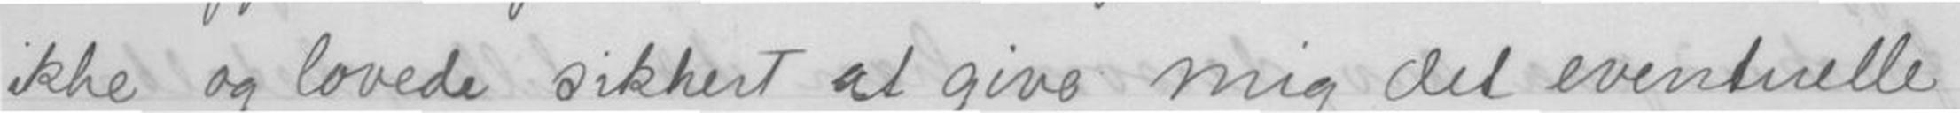ikke og lovede sikkert at give mig det eventuelle
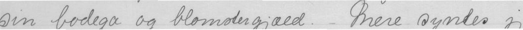sin bodega og blomstergjæld. _ Mere syntes jeg
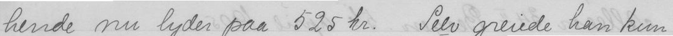hende nu lyder paa 525 kr. Selv greiede han kun
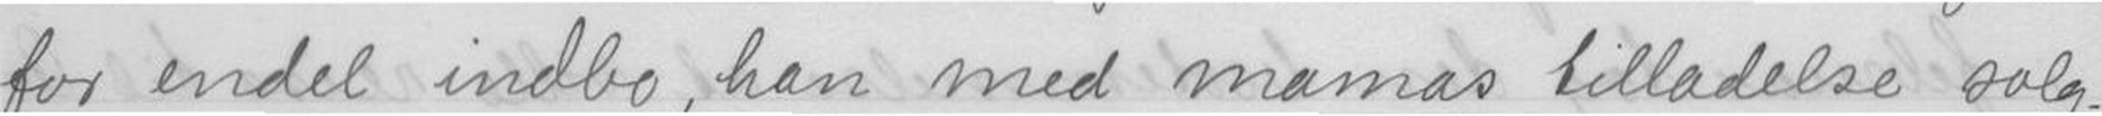for endel indbo, han med mamas tilladelse solg-
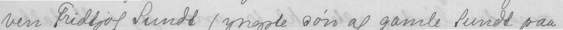ven Fritdjof Sundt (yngste søn af gamle Sundt paa
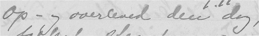Op - og overleved den dag,
